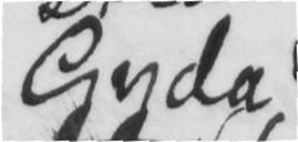Gyda
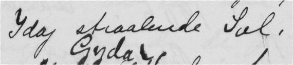Idag straalende Sol.
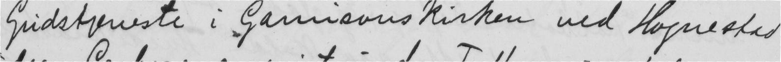Gudstjeneste i Garnisonskirken ved Hognestad
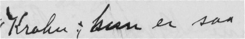Krohn; hun er saa
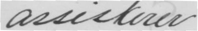assisterer.
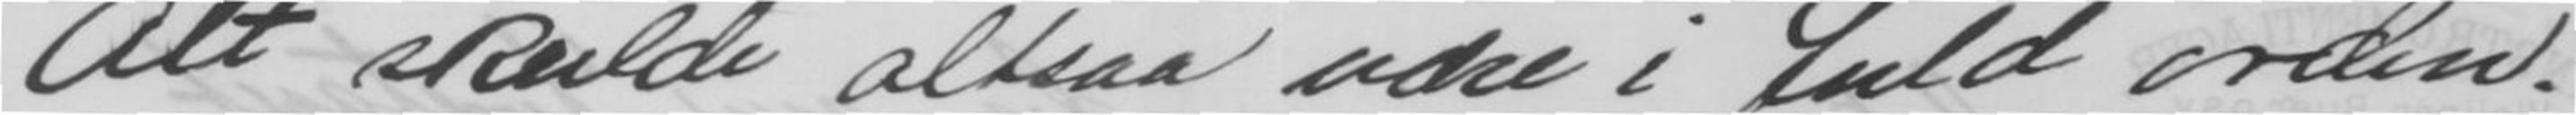Alt skulde altsaa være i fuld orden.
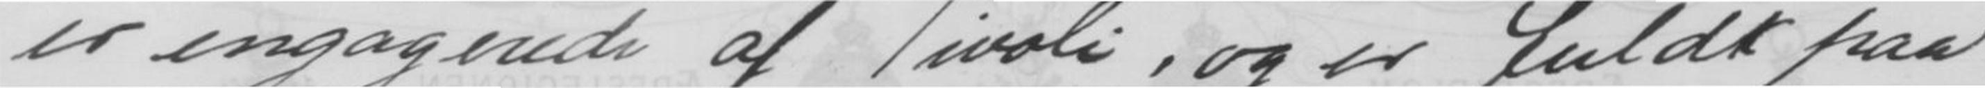er engagerede af Tivoli, og er fuldt paa
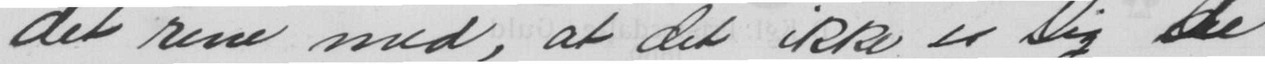det rene med, at det ikke er Dig De
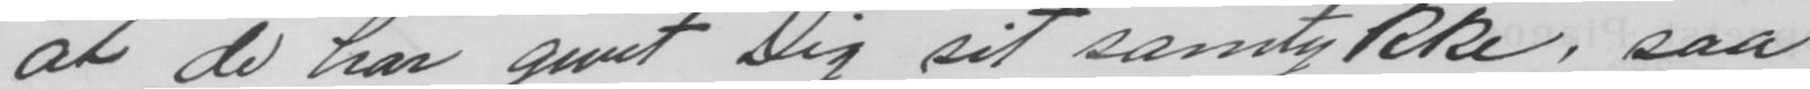at de har givet Dig sit samtykke, saa
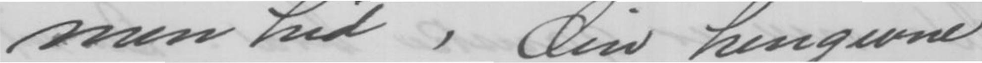men hid, din hengivne
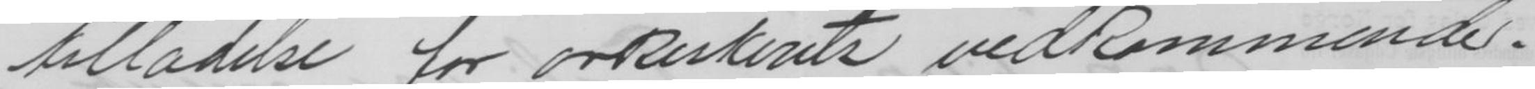tilladelse for orkesterets vedkommende.
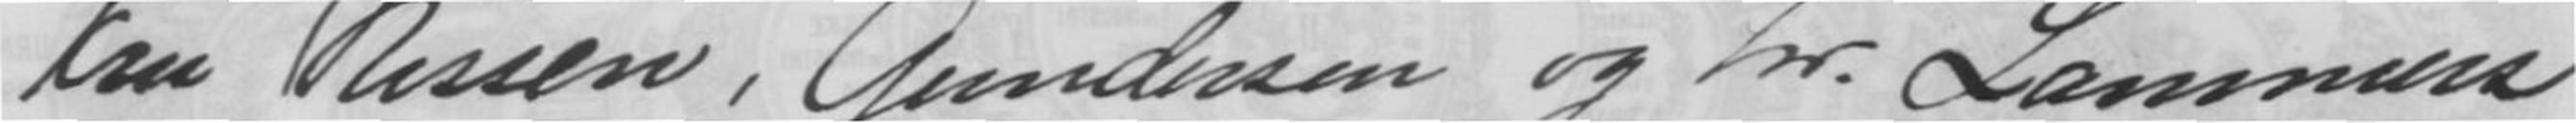Fru Nissen, Gundersen og hr Lammers
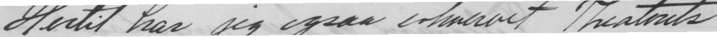Hertil har jeg ogsaa erhvervet Theatrets
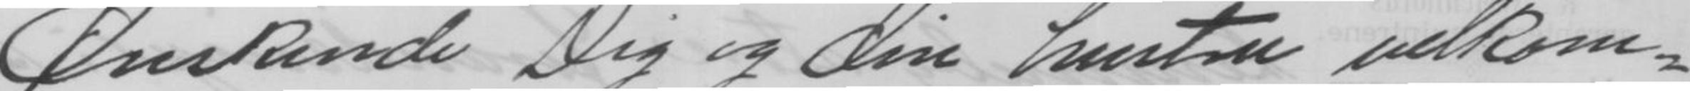Ønskende Dig og din hudtru velkom-
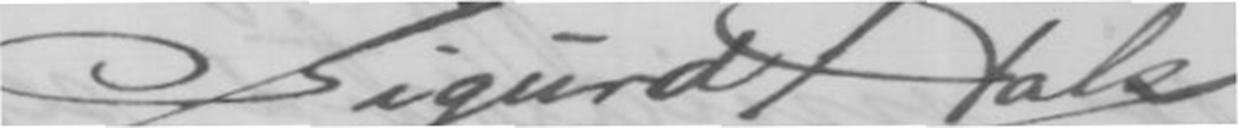Sigurd Hals
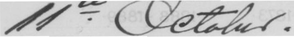11te October.
